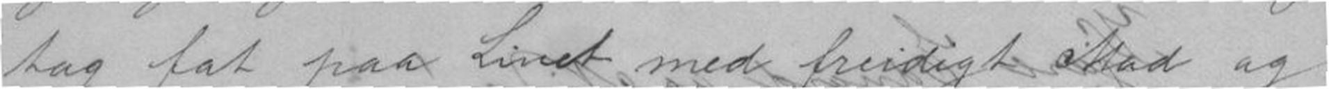tag fat paa Livet med freidigt Mod og
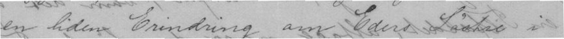en liden Erindring om Eders Søster i
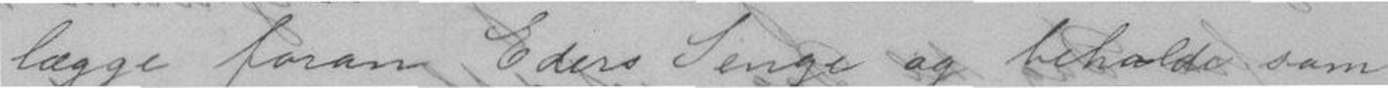lægge foran Eders Senge og beholde som
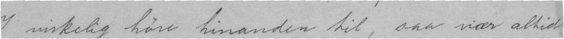I virkelig høre hinanden til, saa vær altid
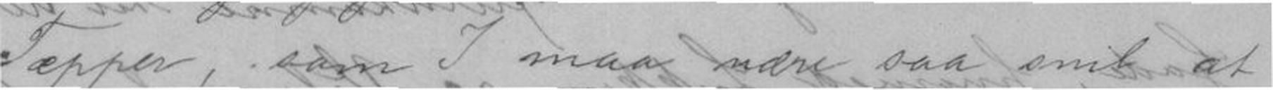Tæpper, som I maa være saa snil at
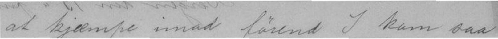at kjæmpe imod førens I kom saa
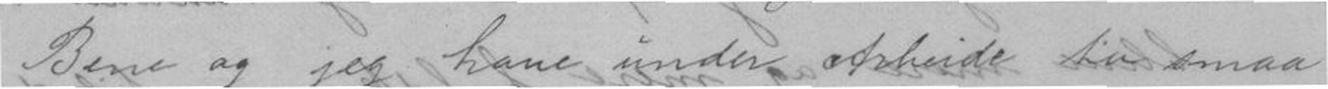Bene og jeg have under Arbeide to smaa
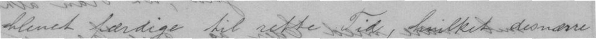blevet færdige til rette Tid, hvilket desværre
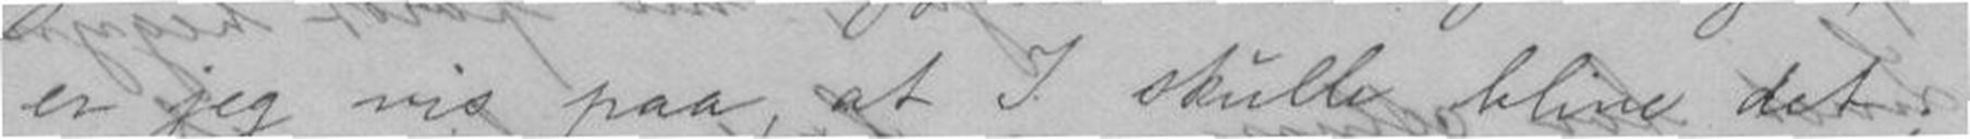er jeg vis paa, at I skulle blive det;
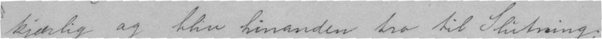kjærlig og bliv hinanden tro til Slutning;
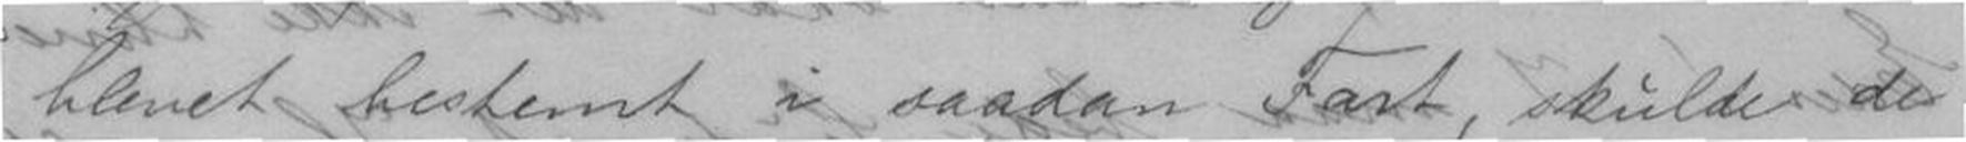blevet bestemt i saadan Fart, skulde der
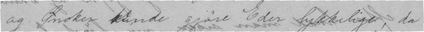og ønsker kunde gjøre Eder lykkelige; da
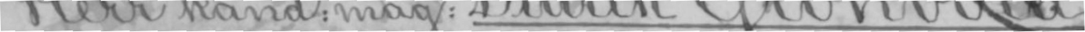Herr kand: mag: Didrik Grønvold.
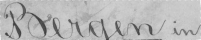Bergen in
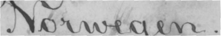Norwegen.
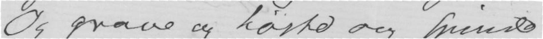Og grave og høste og spinde
